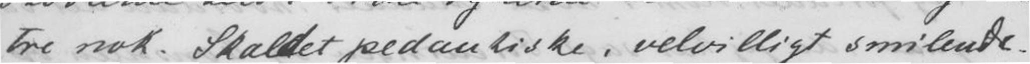tre nok. Skaldet pedantiske, velvilligt smilende.
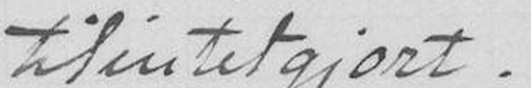tilintetgjort.
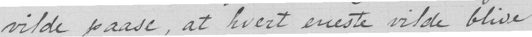vilde paase, at hvert eneste vilde blive
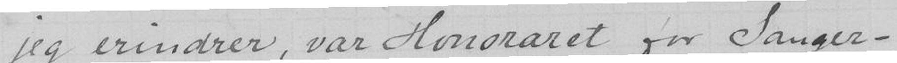jeg erindrer, var Honoraret før Sanger-
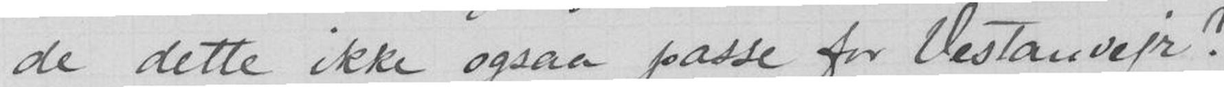de dette ikke ogsaa passe for Vestanvejr.!
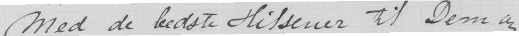Med de bedste Hilsener til Dem og
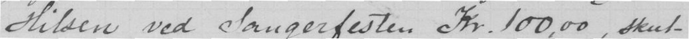Hilsen ved Sangerfesten Kr. 100.00, skul-
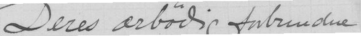Deres ærbøfig forbundne
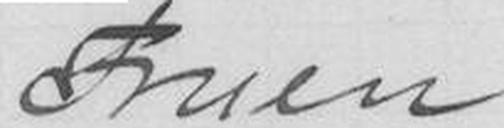Fruen
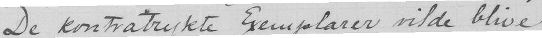De kontratrykte Exemplarer vilde blive
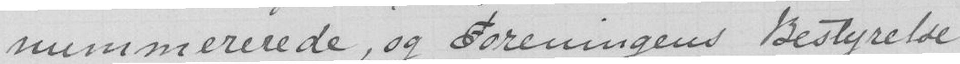nummererede, og Foreningens Bestyrlse
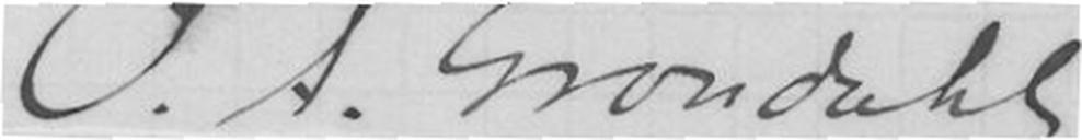O.A. Grøndahl
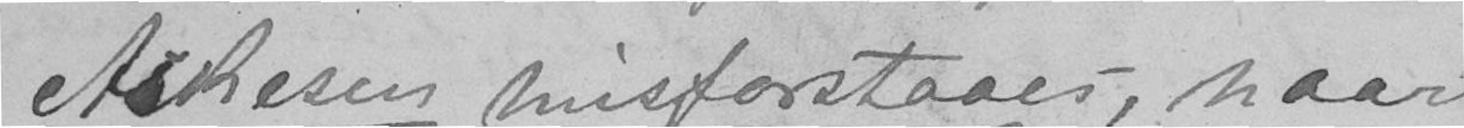Askesen misforstaaes, naar
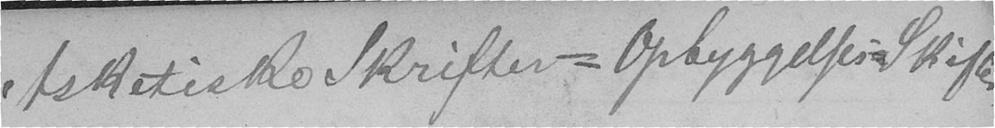Asketiske Skrifter = Opbyggelses -Skrifter
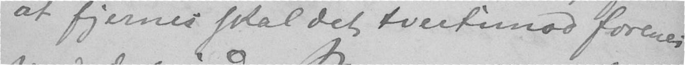at fjernes skal det tvertimod forenes
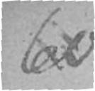60
